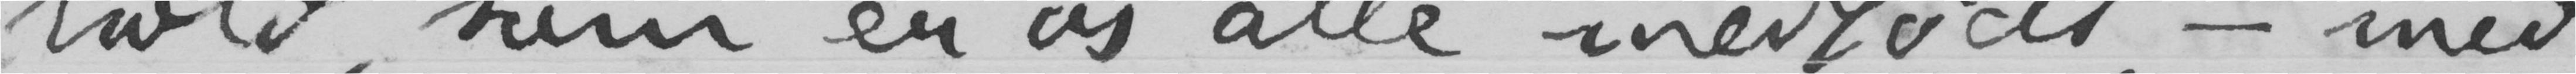hold, som er os alle medfødt, – med
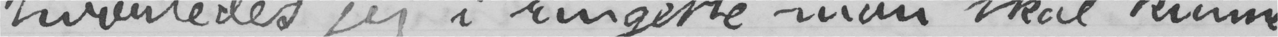hvorledes jeg i ringeste mon skal kunne
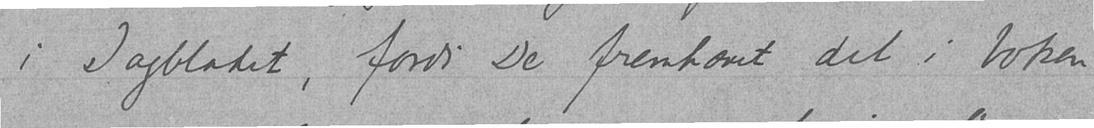i Dagbladet, fordi De fremhævet det i boken
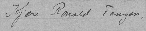Kjære Ronald Fangen,
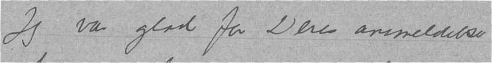Jeg var glad for Deres anmeldelse
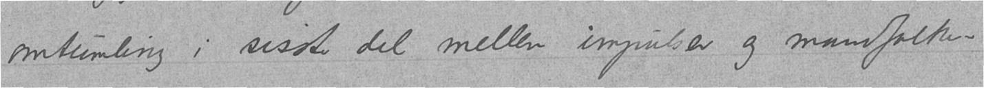omtumling i sisste del mellem impulser og mandfolk-
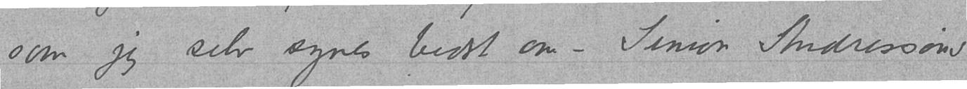som jeg selv synes bedst om – Simon Andressøns
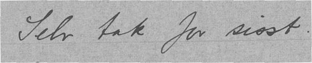Selv tak for sisst.
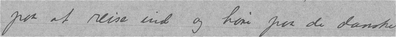paa at reise ind og høre paa de danske
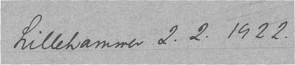Lillehammer 2.2.1922
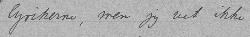lyrikerne, men jeg vet ikke
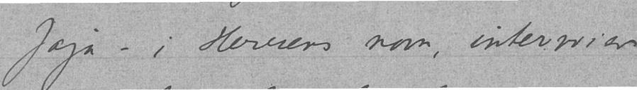Jaja – i Herrens navn, interview
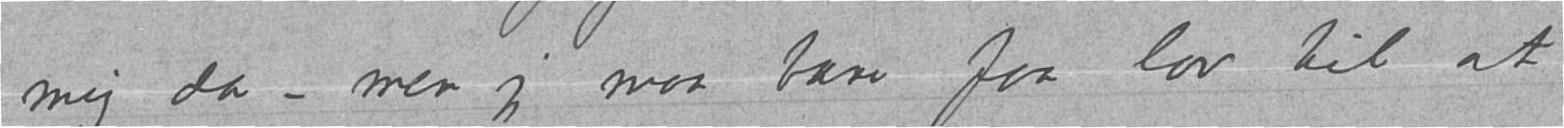mig da – men jeg maa bare faa lov til at
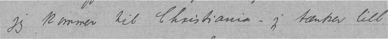jeg kommer til Christiania – jeg tænker litt
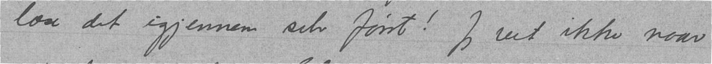læse det igjennem selv først! Jeg vet ikke, naar
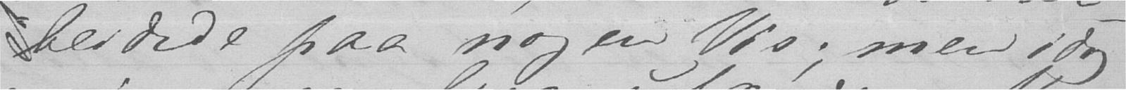beidede paa nogen Vis; men idag
